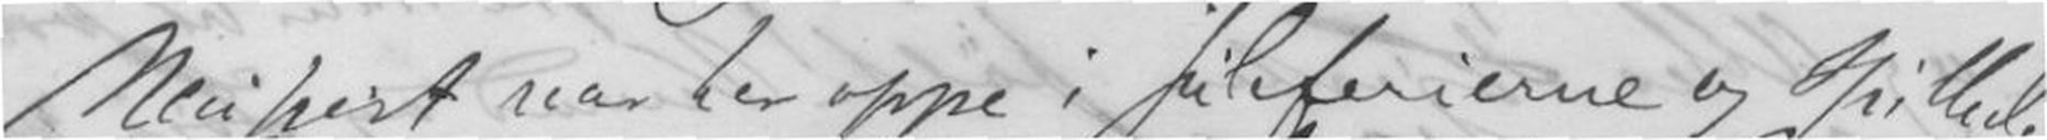Neupert var her oppe i Juleferierne og spillede
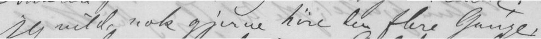jeg vilde nok gjerne høre den flere Gange;
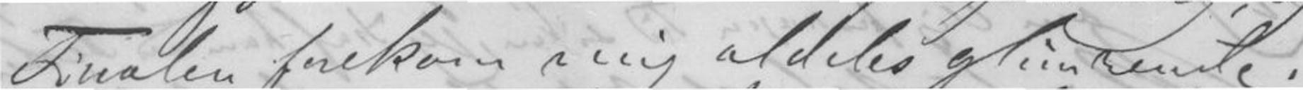Finalen forekom mig aldeles glimrende;
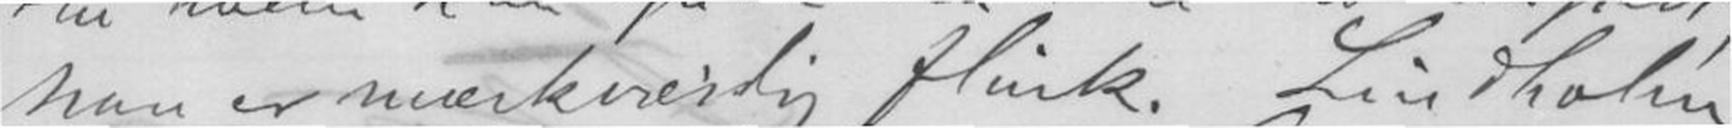han er mærkværdig flink. Lindholm
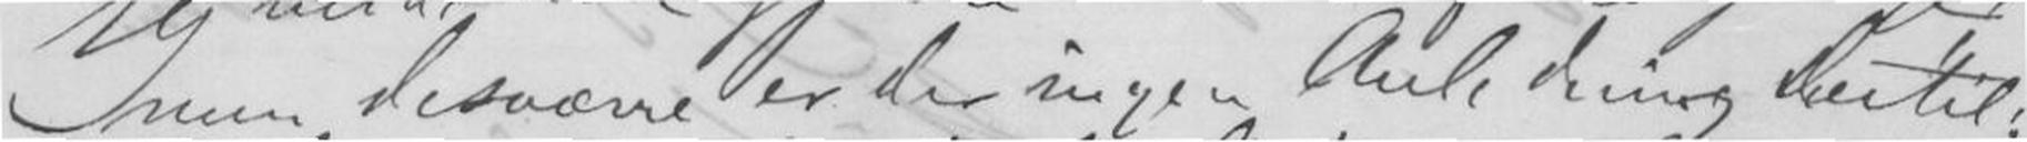men desværre er der ingen Anledning dertil;
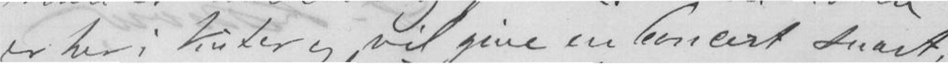er her i Vinter og vil give en Concert snart;
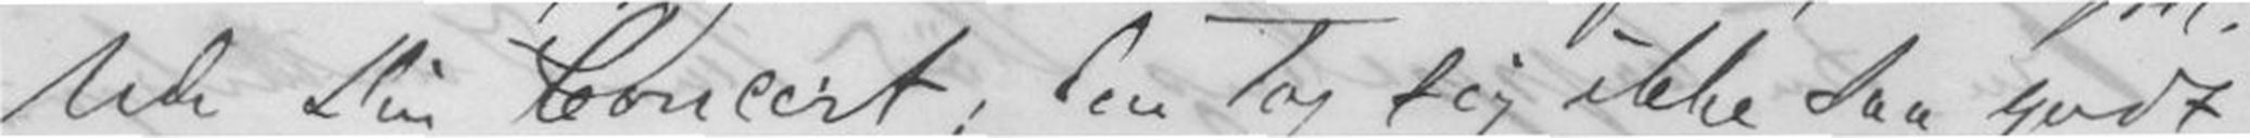lede Din Concert; den Tog sig ikke saa godt
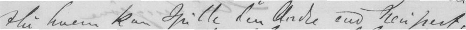thi hvem kan spille den Andre end Neupert;
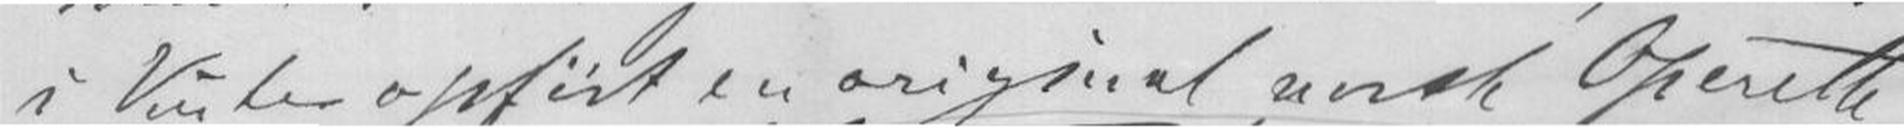i Vinter opført en original norsk Oprette,
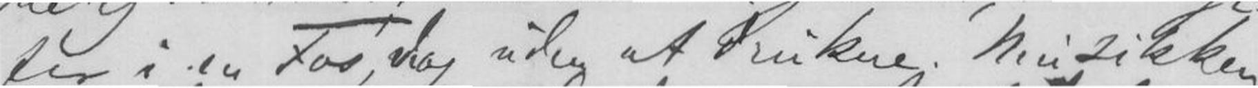ter i en Fos, dog uden at drukne. Musikken
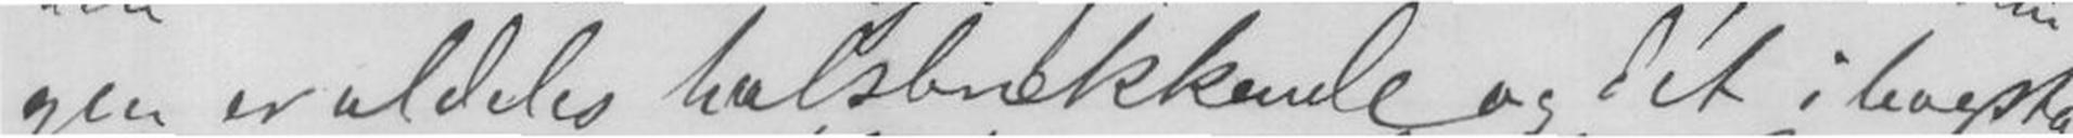gen er aldeles halsbrekkende og det i bogsta-
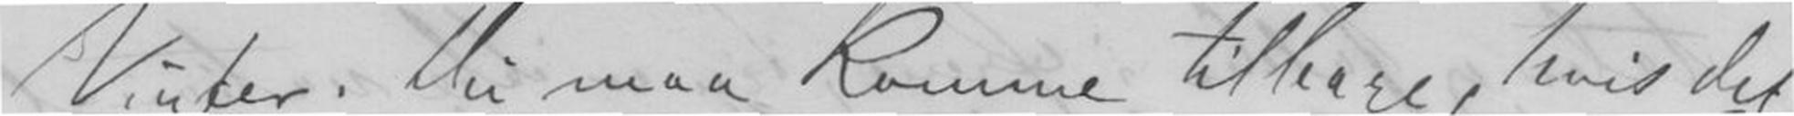Vinter; Du maa komme tilbage, hvis det
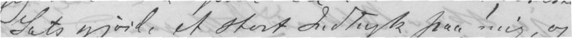Sats gjorde et stort Indtryk paa mig, og
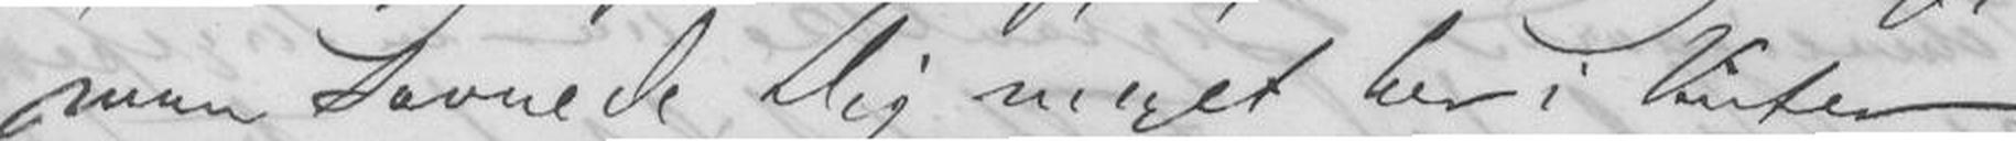man savnede Dig meget her i Vinter
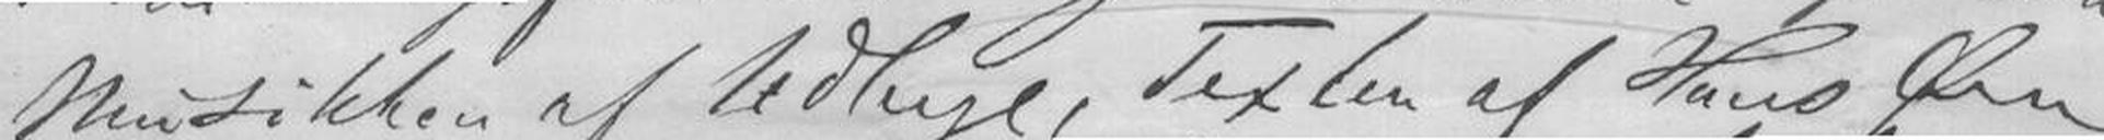Musikken af Udbye, Texten af Hans Øen
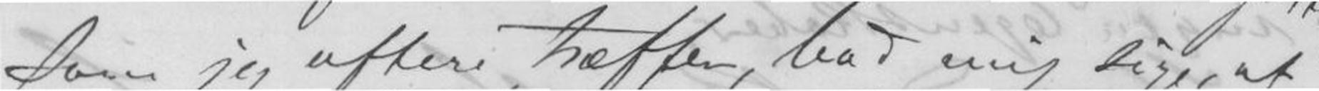som jeg oftere træffer, bad mig sige, at
